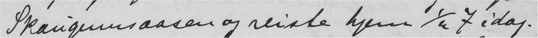Skaugennsaasen og reiste hjem 1/4 7 idag.
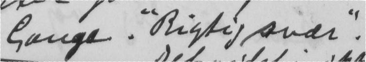Gange. "Rigtig svær".
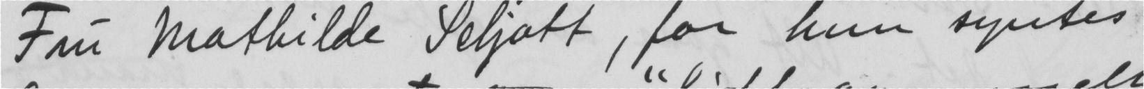Fru Mathilde Schjøtt, for hun syntes
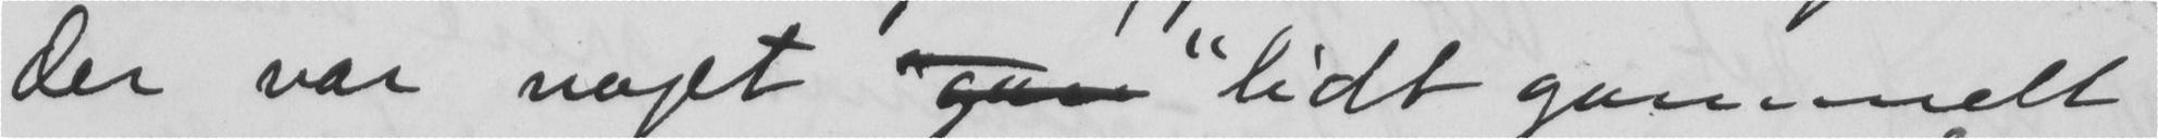der var noget  "lidt gammelt
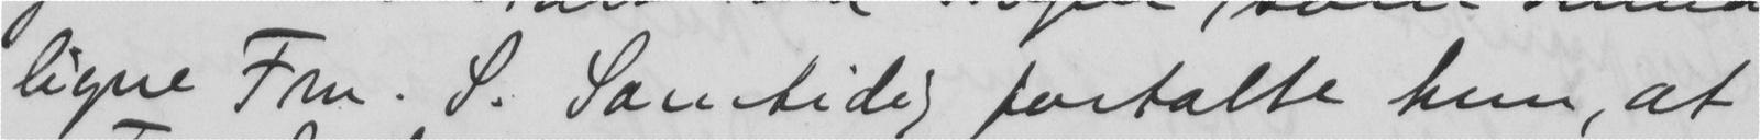ligne Fru. S. Samtidig fortalte hun, at
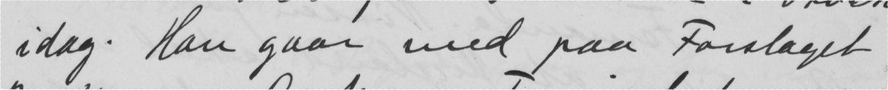idag. Han gaar med paa Forslaget
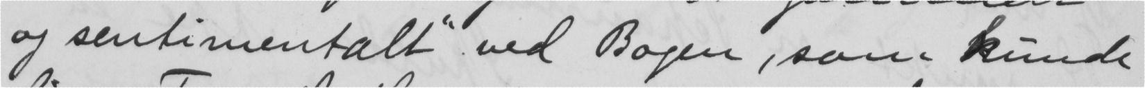og sentimentalt" ved Bogen, som kunde
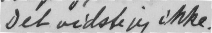Det vidste jeg ikke.
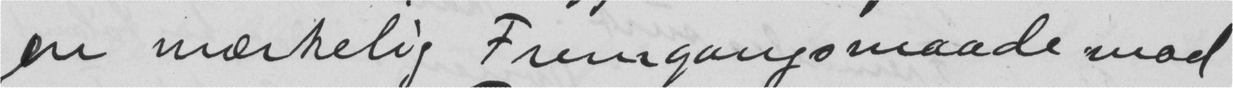en mærkelig Fremgangsmaade mod
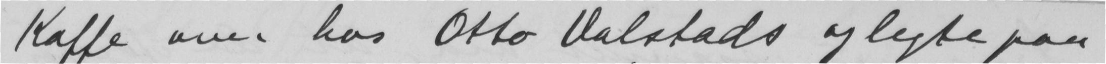Kaffe over hos Otto Valstads og legte paa
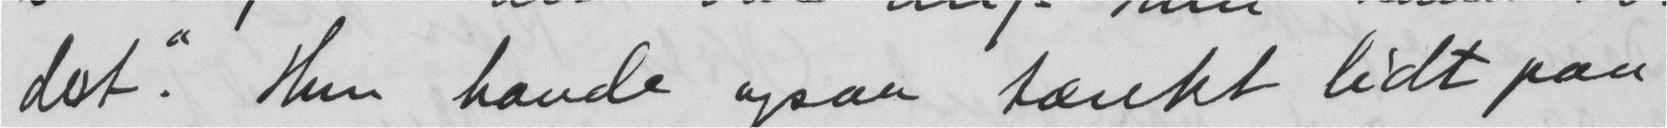det". Hun havde ogsaa tænkt lidt paa
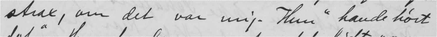strax, om det var mig. Hun "havde hørt
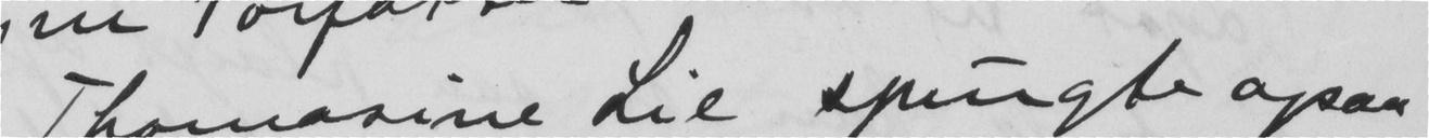Thomasine Lie spurgte ogsaa
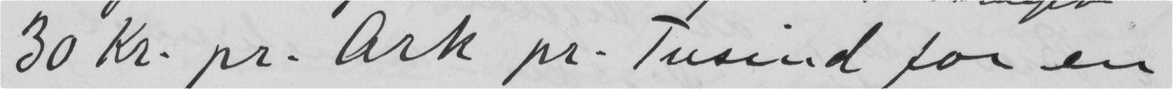30 Kr. pr.  Ark pr. Tusind for en
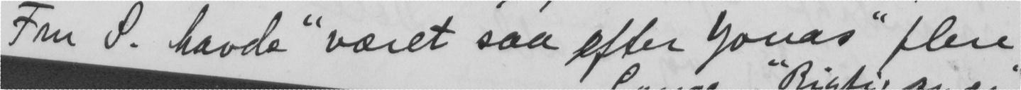Fru S. havde "været saa efter Jonas" flere
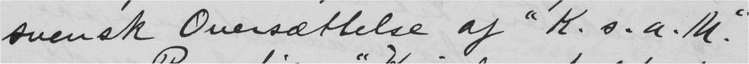svensk Oversættelse af "K. s. a. M."
